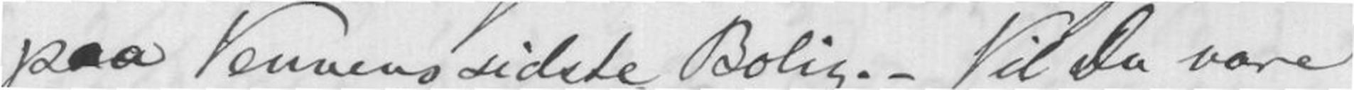paa Vennens sidste Bolig. - Vil Du være
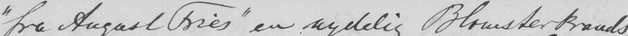"fra August Fries" en nydelig Blomsterkrands -
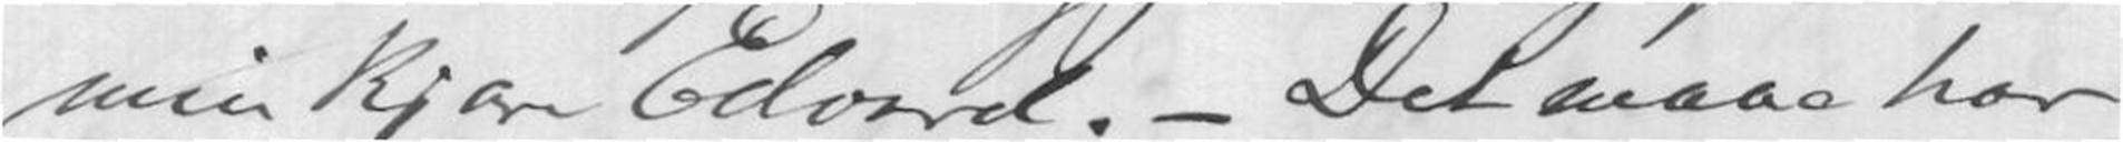min kjære Edvard. - Det maae har
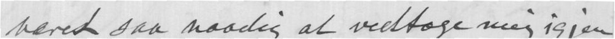været saa naadig at vedtage mig igjen
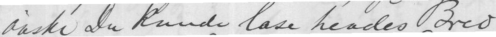ønske Du kunde læse hendes Brev
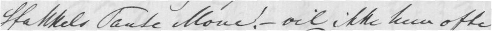Stakkels Tante Mone! - vil ikke hun ofte
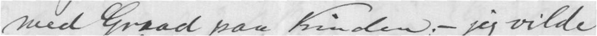med Graad paa Kinden; - jeg vilde
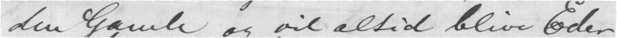den Gamle og vil altid blive Eder
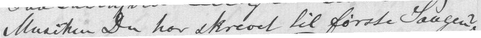Musiken Du har skrevet til førdte Sangen?
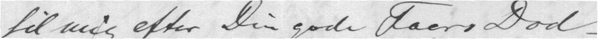til mig efter Din gode Faers Død -
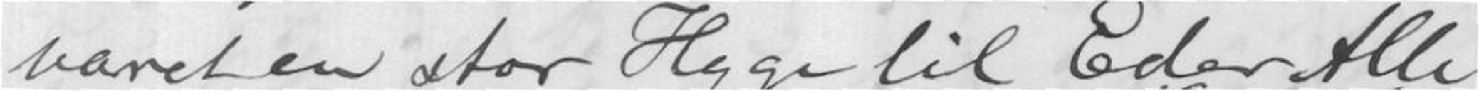varet en stor Hyge til Eder Alle
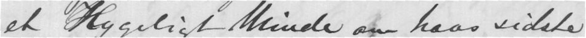et Hygeligt Minde om hans sidste
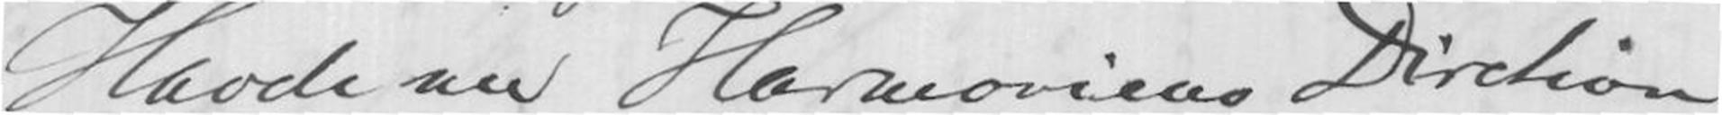Havde nu Harmoniens Diretion
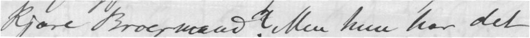kjære Broermand? Men hun har det
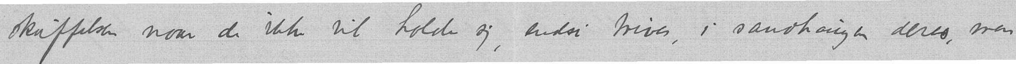skuffelsen naar de ikke vil holde sig, endsi trives, i sandhaugen deres, men
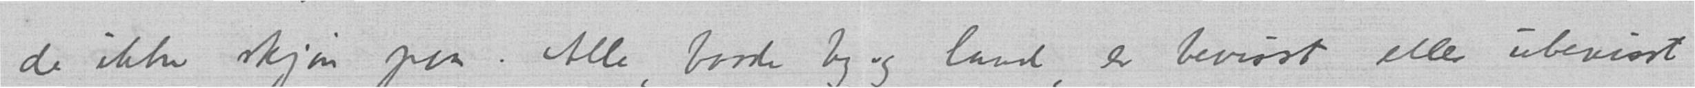de ikke skjøn paa. Alle, baade by og land er bevisst eller ubevisst
